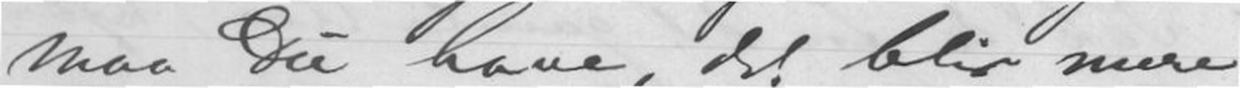maa Du have, det blir mere
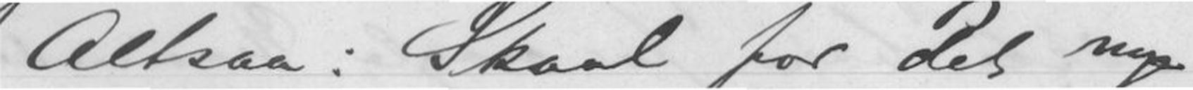Altsaa: skaal for det nye
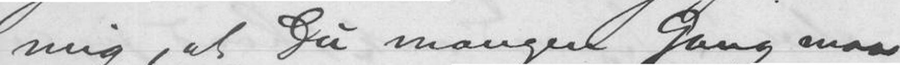mig, at Du mangen Gang maa
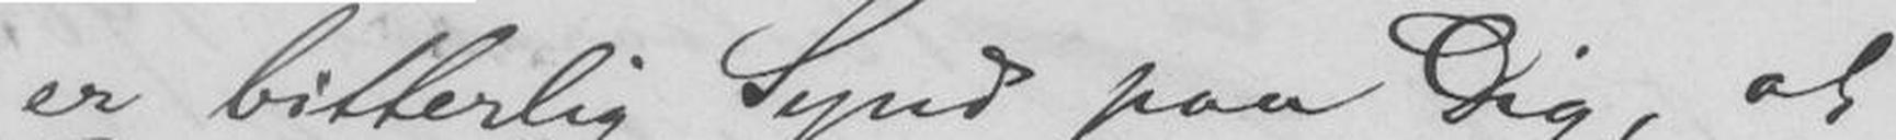er bitterlig Synd paa Dig, at
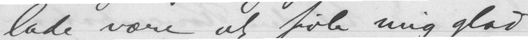lade være at føle mig glad
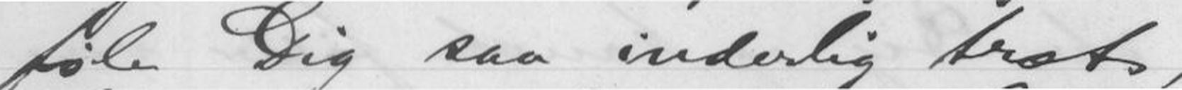føle Dig saa inderlig træt,
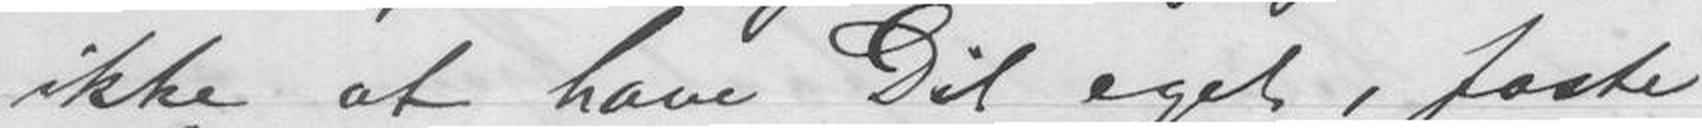ikke at have Dit eget, faste
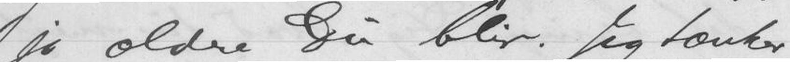jo ældre Du blir. Jeg tænker
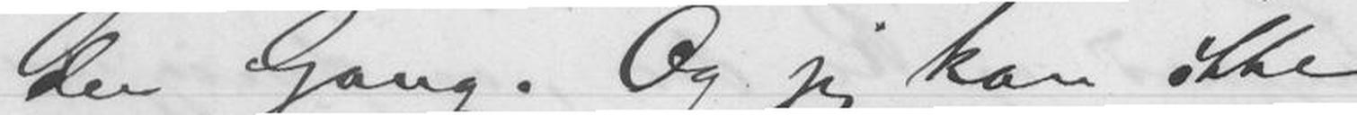den Gang. Og jeg kan ikke
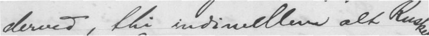derved, thi indimellem alt Rusket
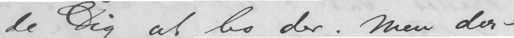de Dig at bo der. Men der-
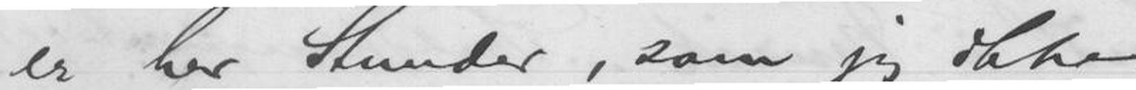er her Stunder, som jeg ikke
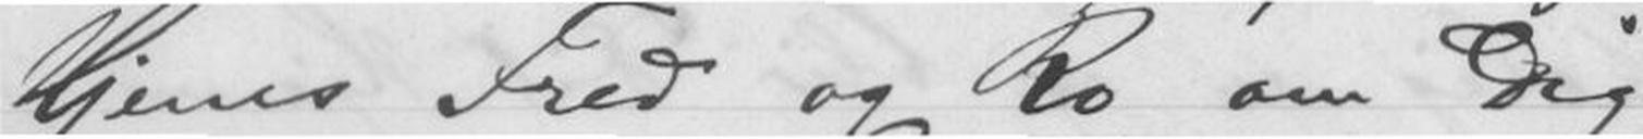Hjems Fred og Ro om Dig.
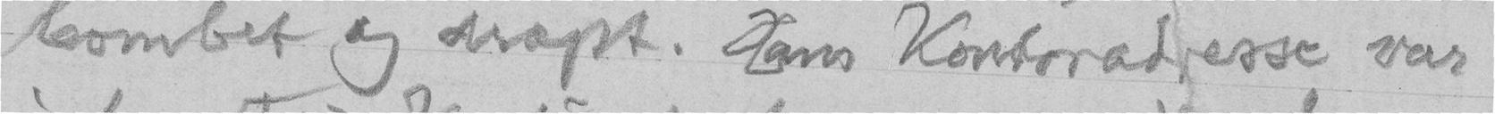bombet og dræpt. Hans Kontoradresse var
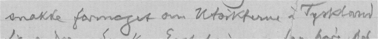snakke formeget om Utsikterne i Tyskland
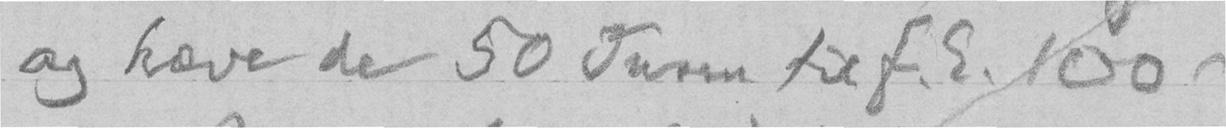og hæve de 50 Tusen til f.E. 100.
